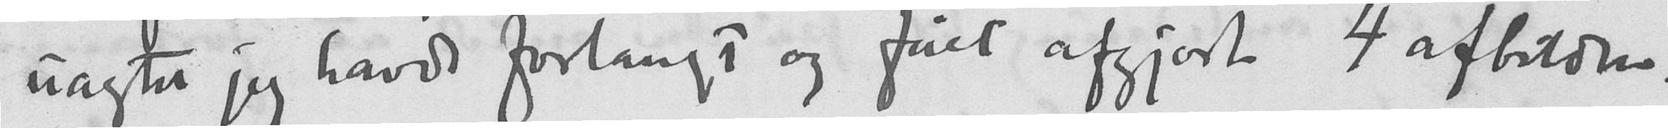uagtet jeg havde forlangt og fået afgjort 4 afbildn.
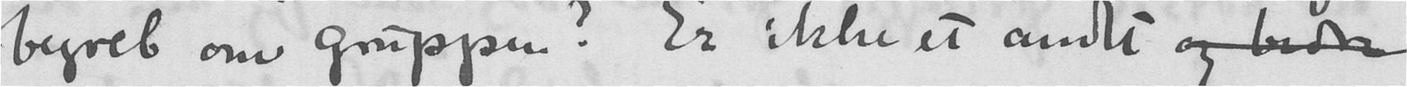begrep om gruppen? Er ikke et andet og bedre
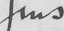Jens
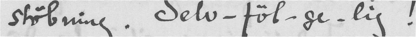støbning. Selv-føl-ge-lig!
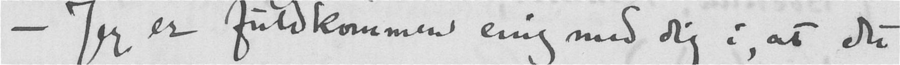- Jeg er fuldkommen enig med dig i, at du
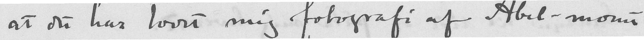at du har lovet mig fotografi af Abel-monu
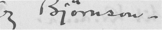Og Bjørnson-
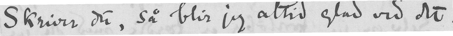Skriver du, så blir jeg altid glad ved det.
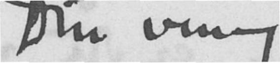Din ven
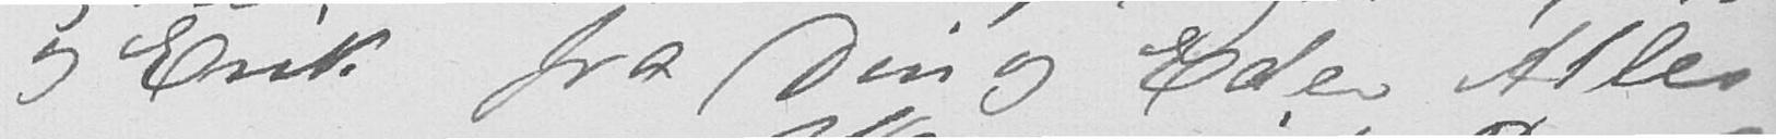og Erik fra Din og Eder Alles
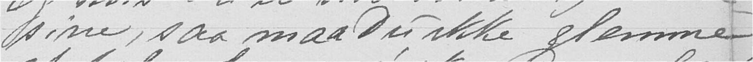sine, saa maa du ikke glemme
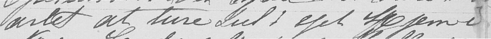artet at ture Jul i eget Hjem i
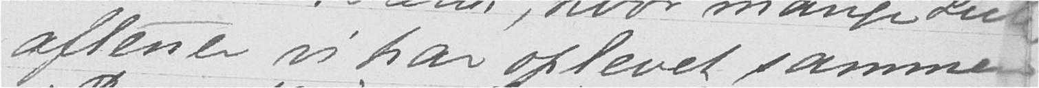aftener vi har oplevet sammen
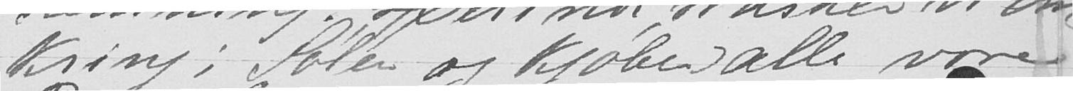kring i Sølen og kjøber alle vore
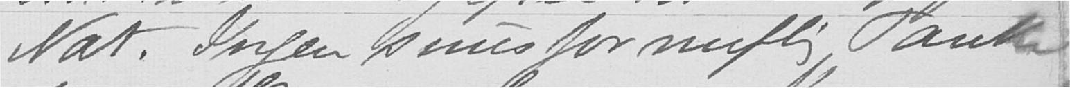Nat. Ingen snusfornuftig Tanke
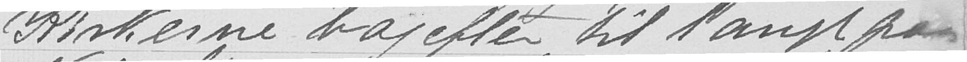Kirkerne bagefter til langt paa
